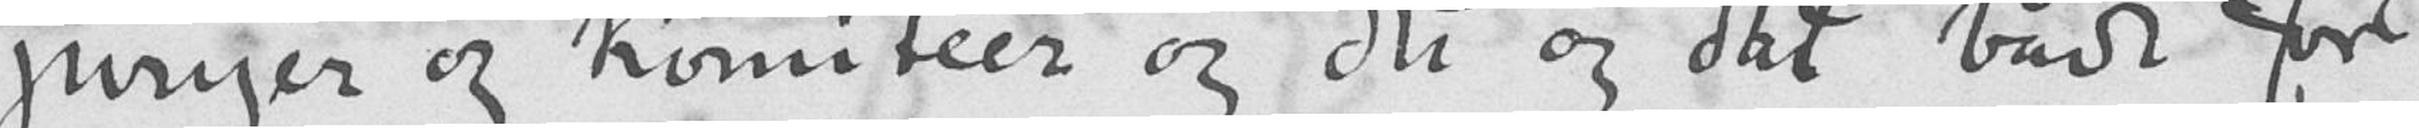juryer og komiteer og dit og dat både for
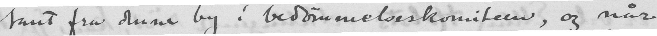tant fra denne by i bedømmelseskomiteen, og når
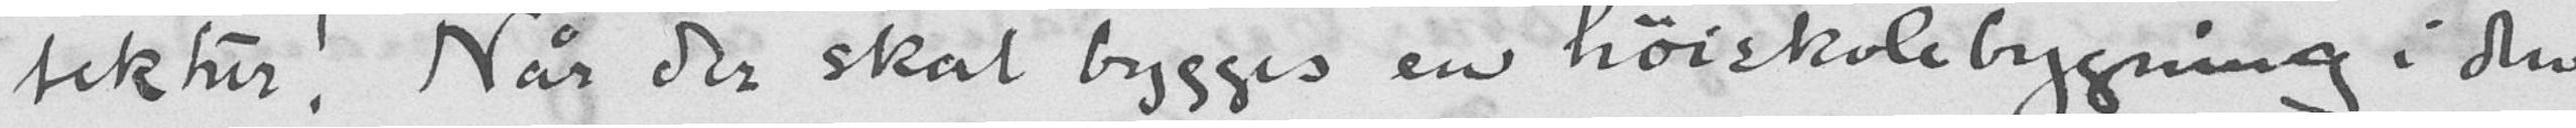tektur! Når der skal bygges en høiskolebygning i den
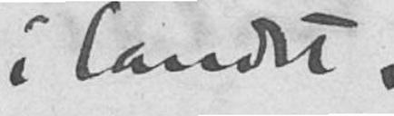i landet.
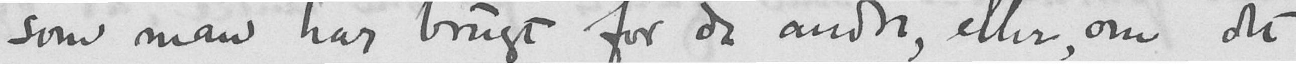som man har brugt for de andre, eller, om det
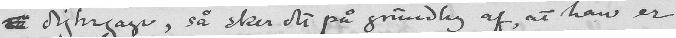"digtergage", så sker det på grundlag af, at han er
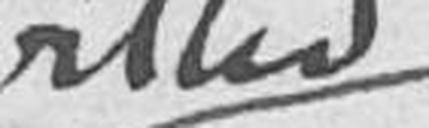billed
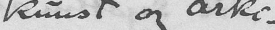kunst og arki-
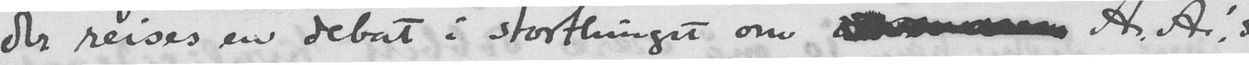der reises en debat i storthinget om A.A.'s
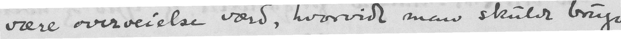vare overveielse værd, hvorvidt man skulde bruge
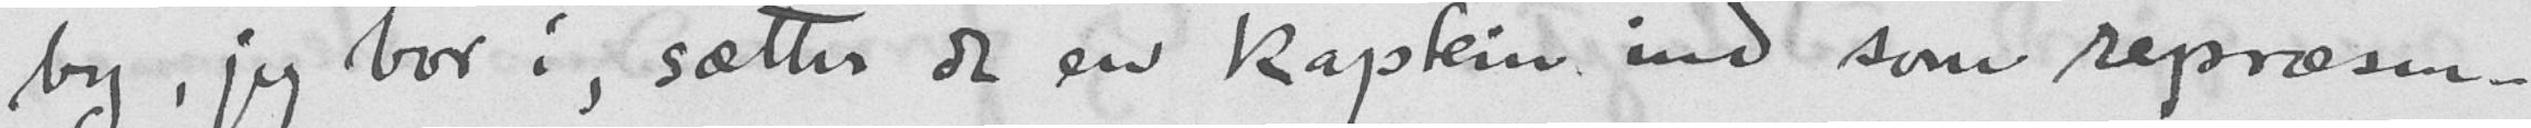by, jeg bor i, sætter de en kaptein ind som repræsen-
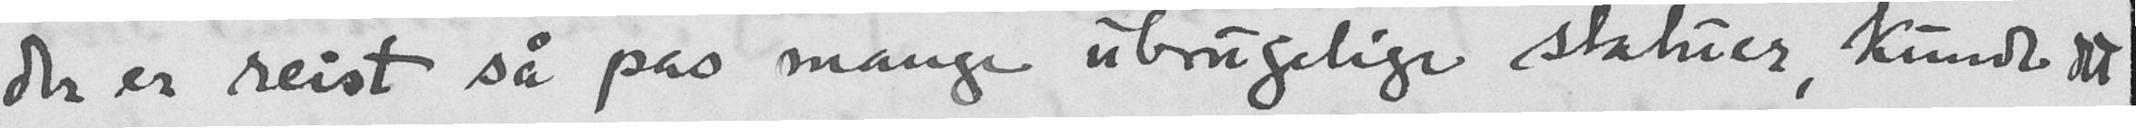der er reist så pas mange ubrugelige statuer, kunde det
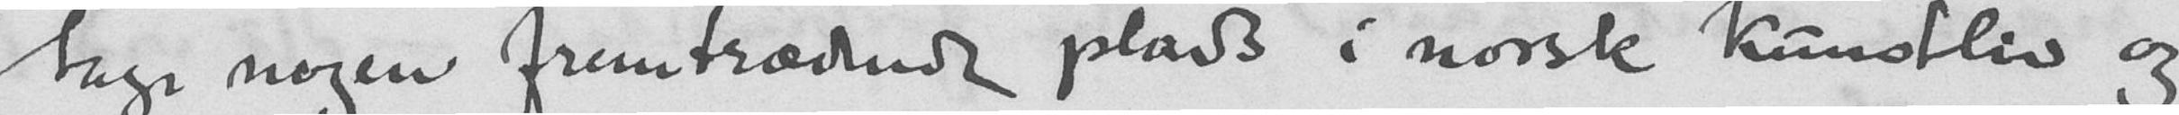tage nogen fremtrædende plads i norsk kunstliv og
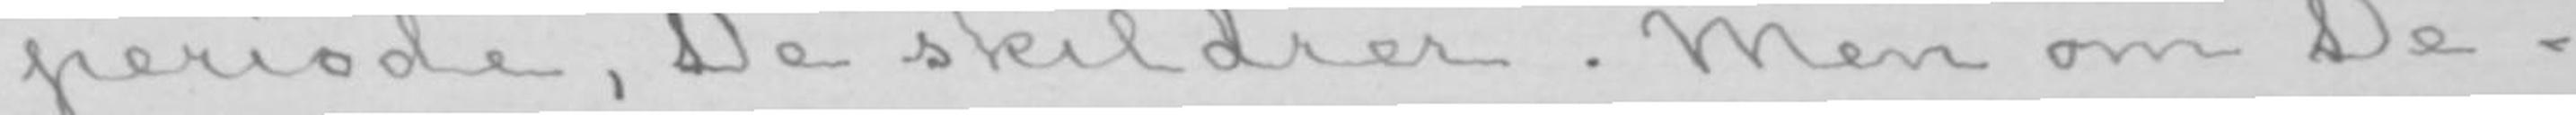periode, De skildrer. Men om De-
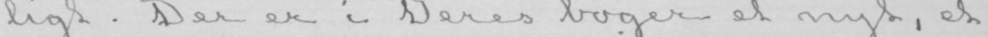ligt. Der er i Deres bøger et nyt, et
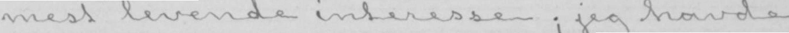mest levende interesse; jeg havde
